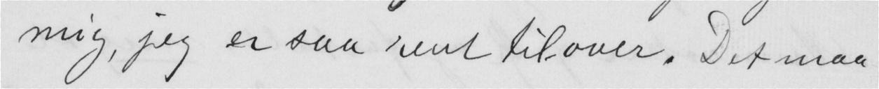mig, jeg er saa rent tilovers. Det maa
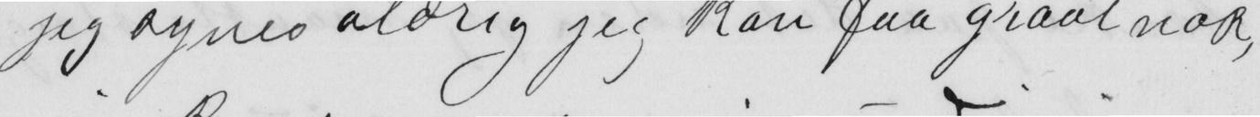jeg synes aldrig jeg kan faa graat nok,
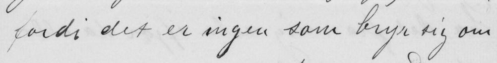fordi det er ingen som bryr sig om
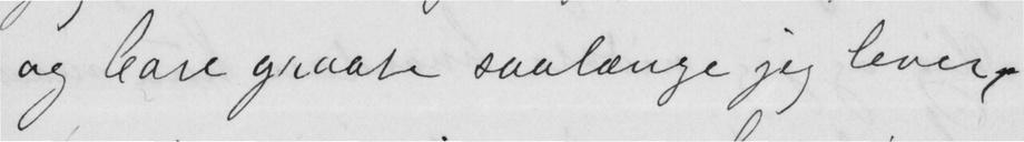og bare graate saalænge jeg lever,
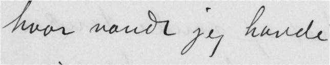hvor vandt jeg havde
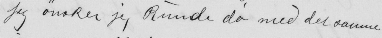jeg ønsker jeg Kunde dø med det samme
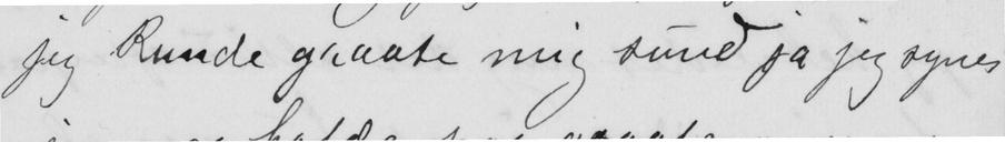jeg Kunde graate mig rund ja jeg synes
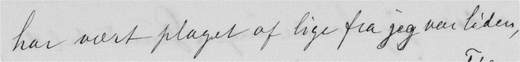har vært plaget af lige fra jeg var liden,
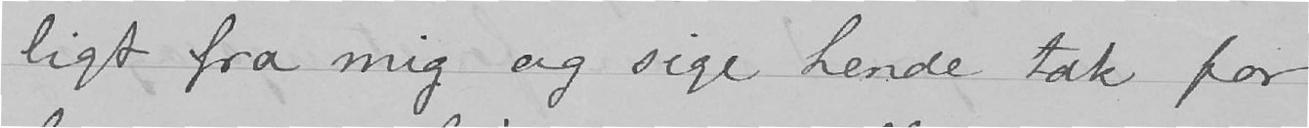ligt fra mig og sige hende tak for
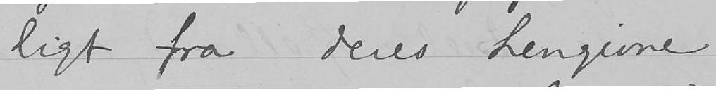ligt fra deres hengivne
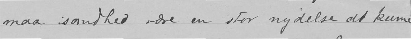maa isandhed være en stor nydelse at kunne
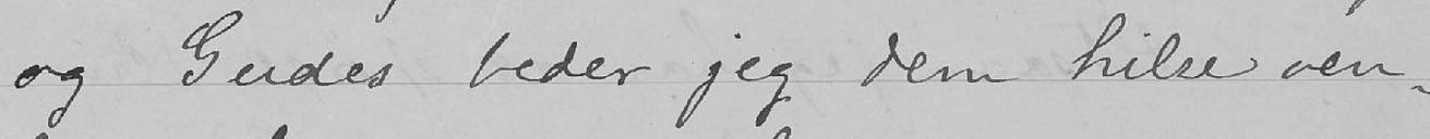og Gudes beder jeg dem hilse ven-
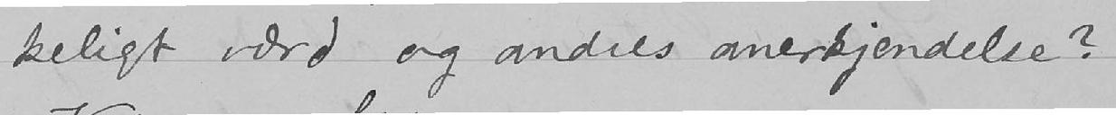keligt værd og andres anerkjendelse?
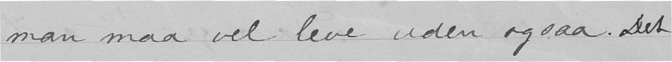man maa vel leve uden ogsaa. Det
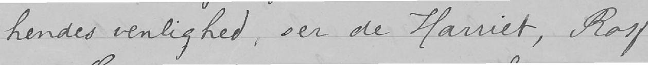hendes venlighed, ser de Harriet, Ross
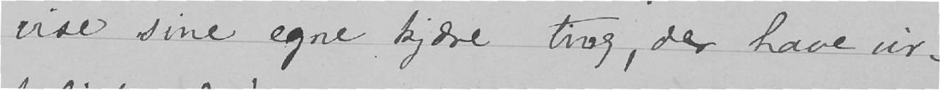vise sine egne kjære ting, der have vir-
